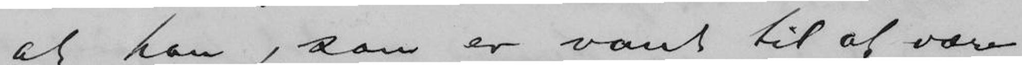at han, som er vant til at være
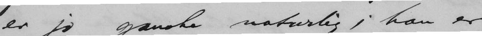er jo ganske naturlig; han er
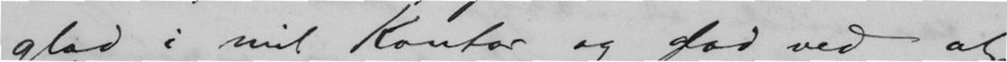glad i mit Kontor og glad ved at
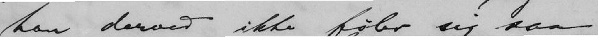han derved ikke føler sig saa
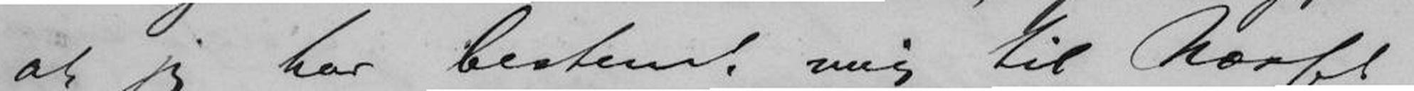at jeg har bestemt mig til Næsset.
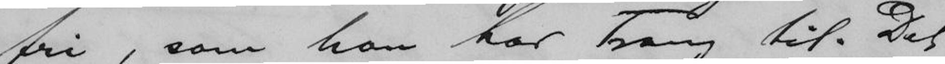fri, som han har Trang til. Det
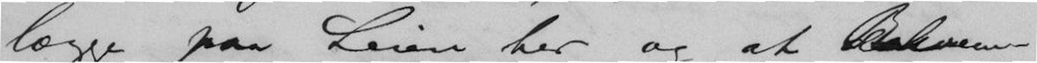lægge paa Leien her og at Bekvem-
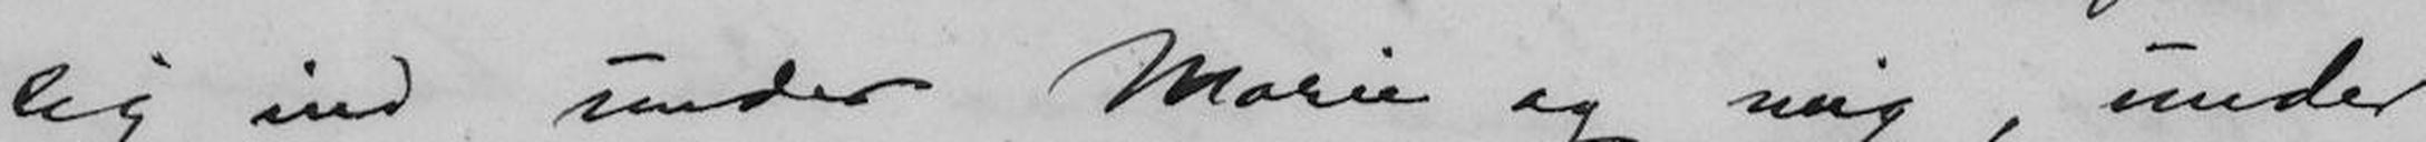sig ind under Marie og mig, under
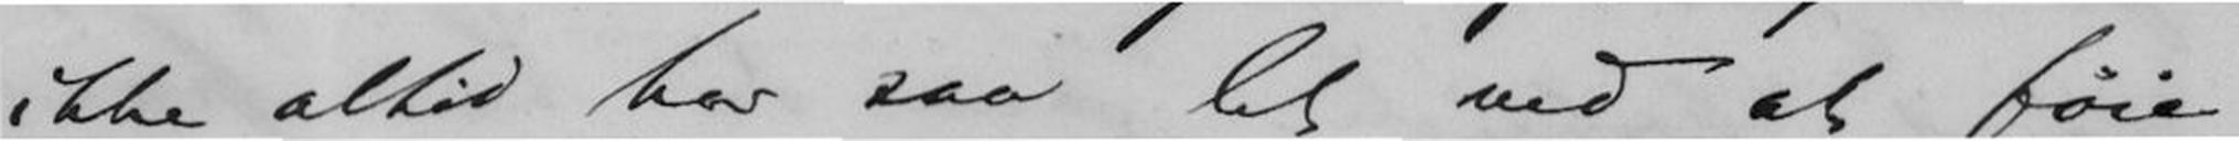ikke altid har saa let ved at føie
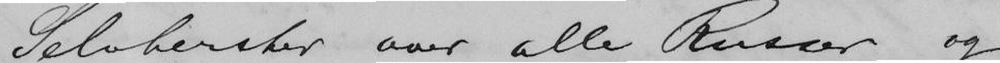Selvhersker over alle Russer og
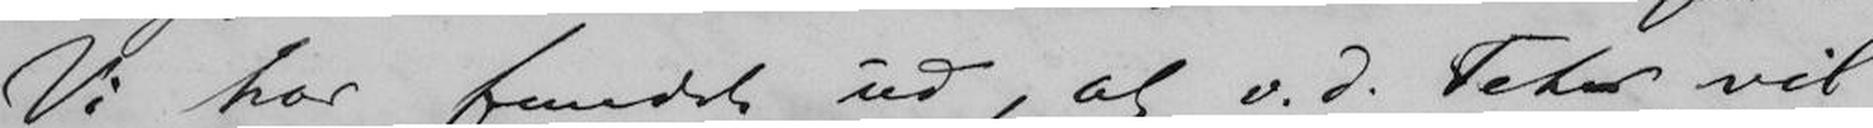Vi har fundet ud, at v.d. Fehr vil
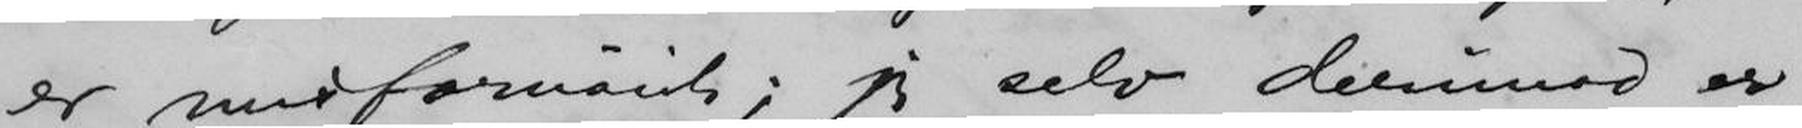er misfornøiet; jeg selv derimod er
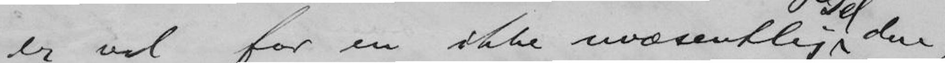er vel for en ikke uvæsentlig den,
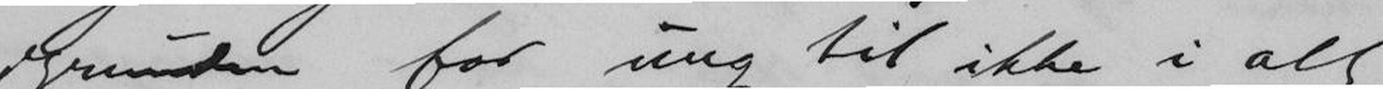igrunden for ung til ikke i alt
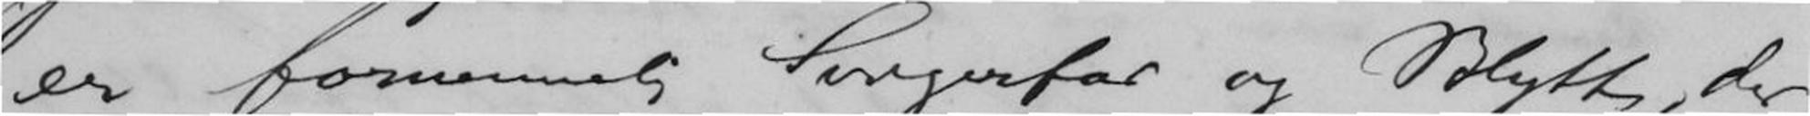er form...elig Svigerfar og Blytt, der
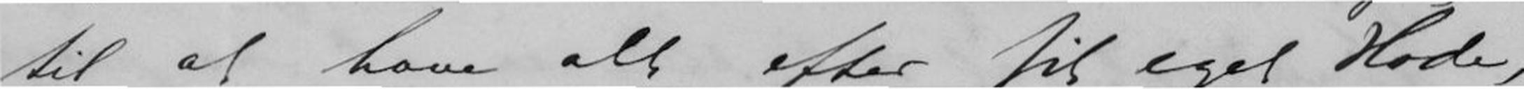til at have alt efter sit eget Hode,
